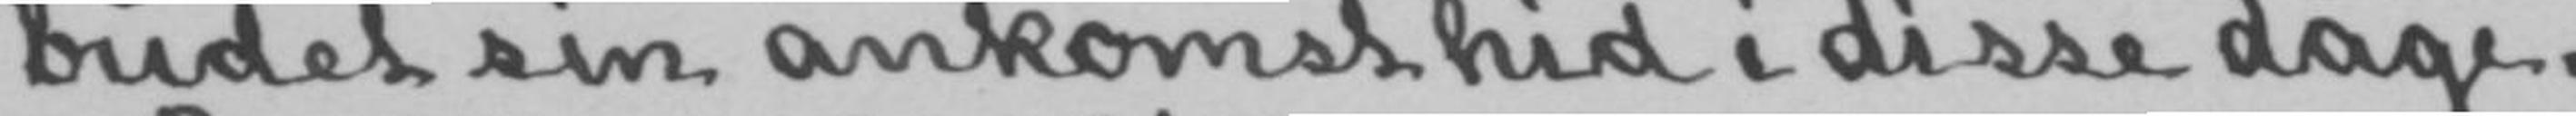budet sin ankomst hid i disse dage.
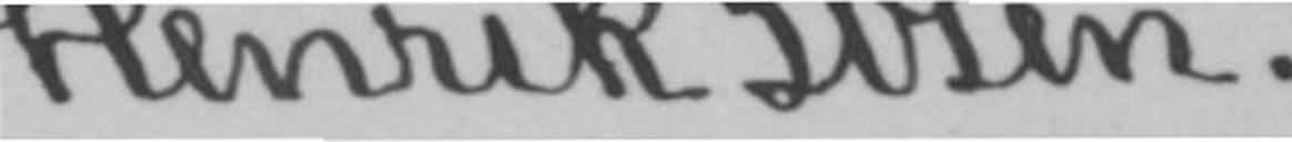Henrik Ibsen.
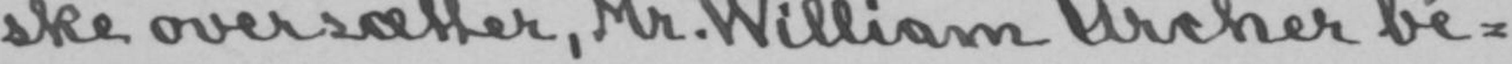ske oversætter, Mr. William Archer be-
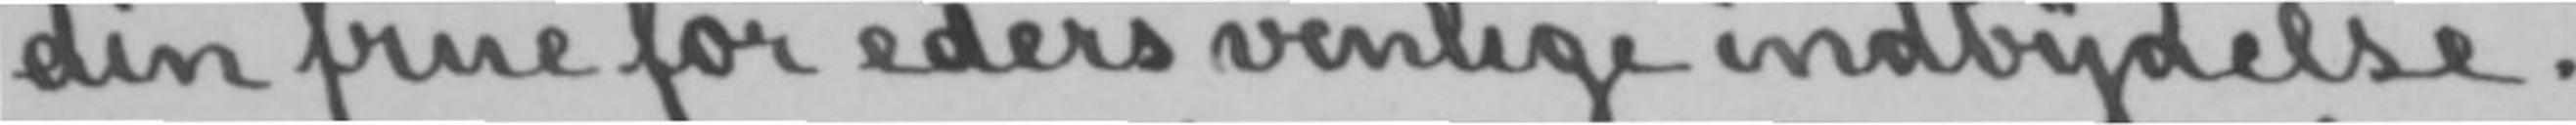din frue for eders venlige indbydelse.
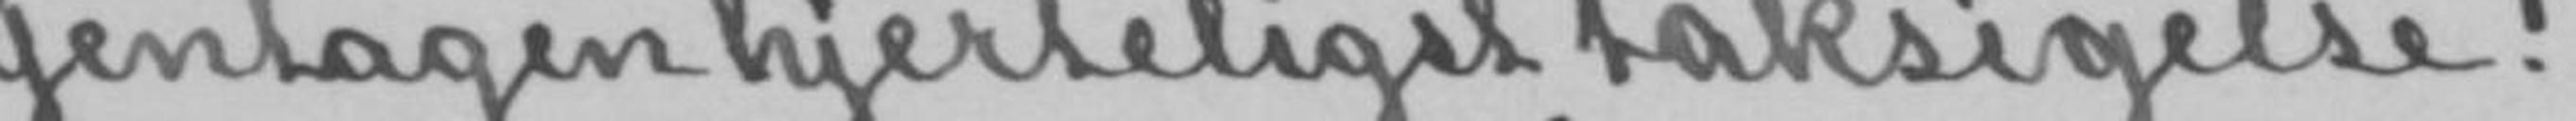Gentagen hjerteligst taksigelse!
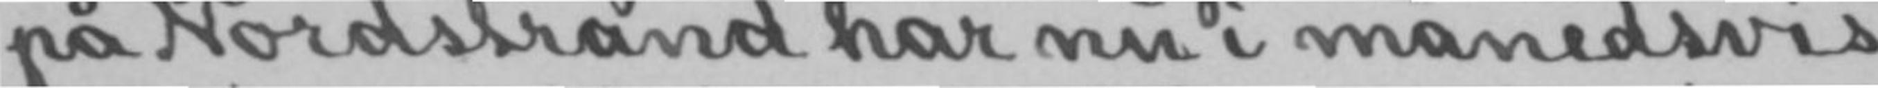på Nordstrand har nu i månedsvis
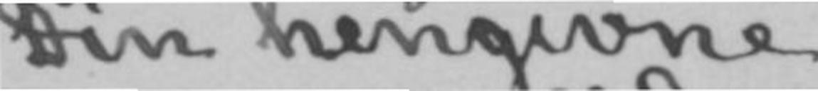Din hengivne
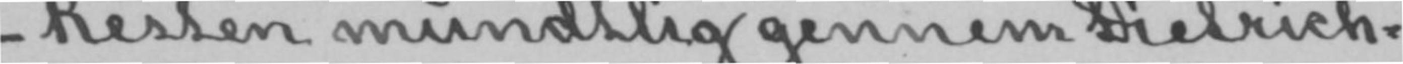- Resten mundtlig gennem Dietrich-
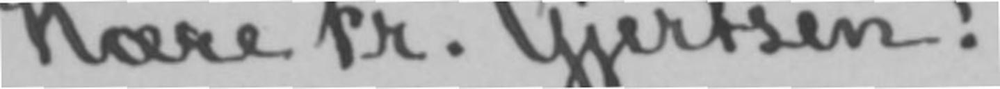Kære Fr. Gjertsen!
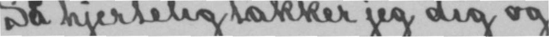Så hjertelig takker jeg dig og
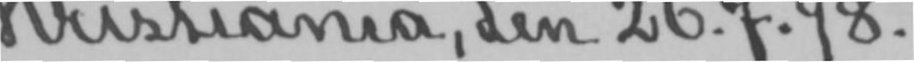Kristiania, den 26.7.98.
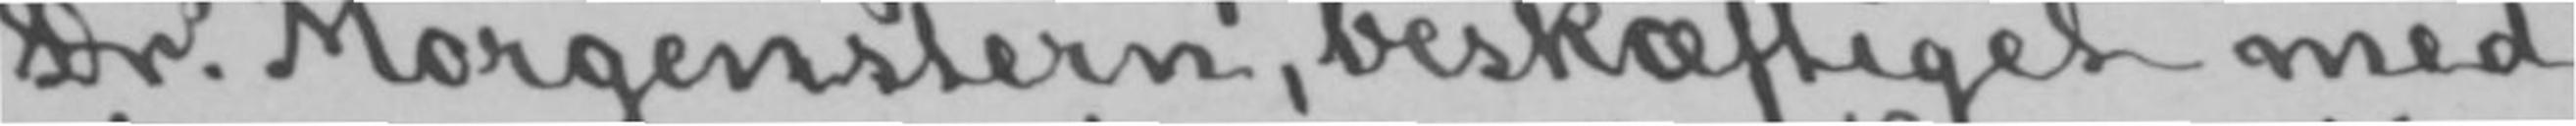Dr. Morgenstern, beskæftiget med
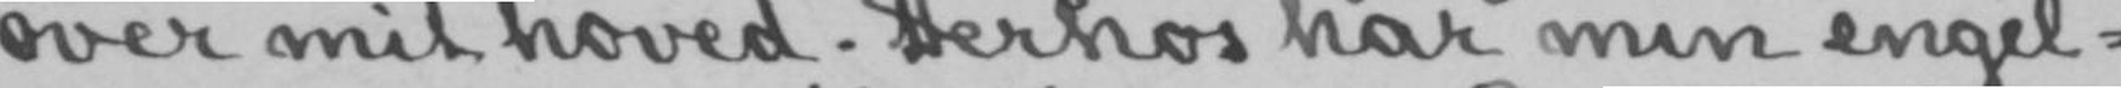over mit hoved. Derhos har min engel-
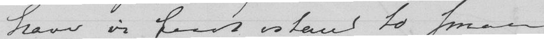haver vi faaet istand to smaa
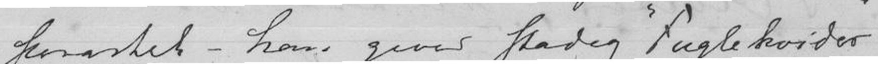storartet - han giver stadig "Fuglekvider"
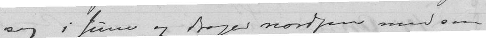sig i Juni og drager nordpaa med sin
